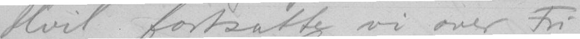Hvil fortsatte vi over Fri-
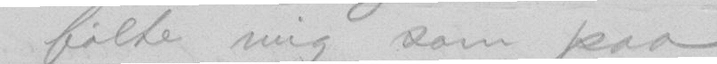Jeg følte mig som paa
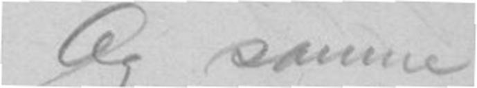Og samme
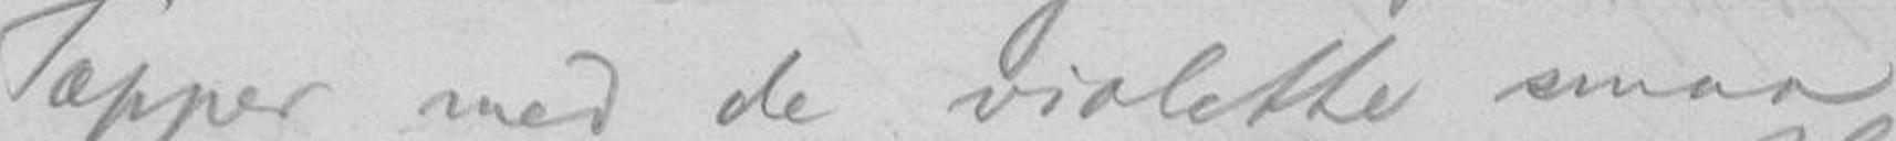Tæpper med de violette smaa
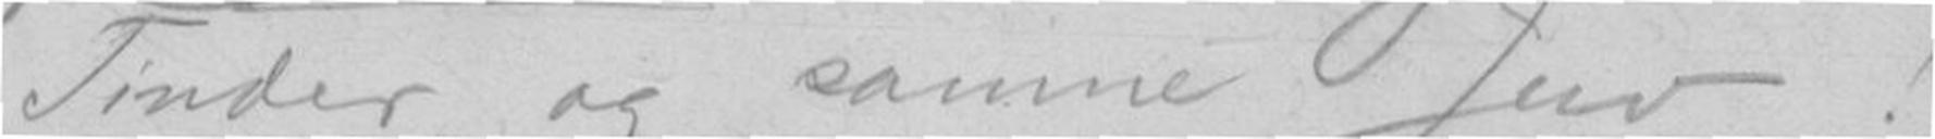Tinder og samme Juv!
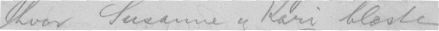hvor Susanne og Kari blæste
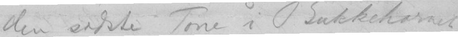den sidste Tone i Bukkehornet
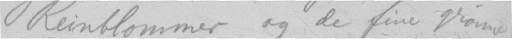Reinblommer og de fine grønne
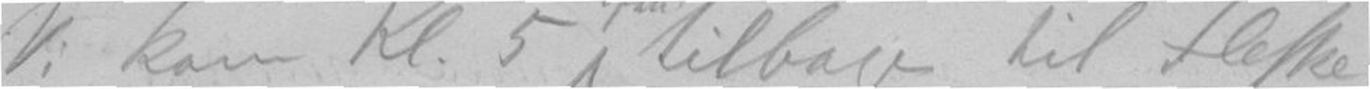Vi kom Kl. 5 tilbage til Flæske-
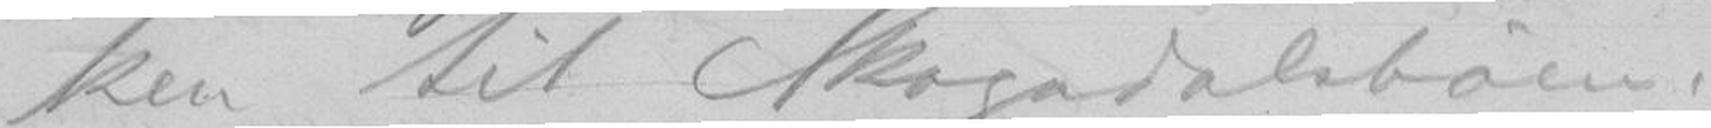ken til Skogadalsbøen.
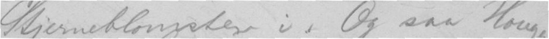Stjerneblomster i. Og saa Hougen,
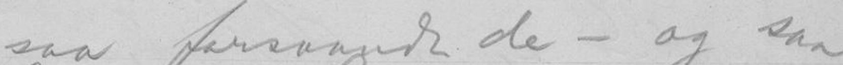- saa forsvandt de - og saa
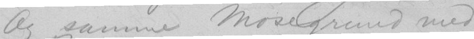Og samme Mosegrund med
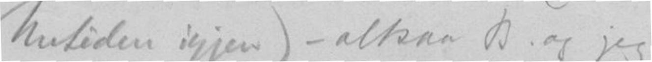Nutiden igjen) - altsaa B. og jeg
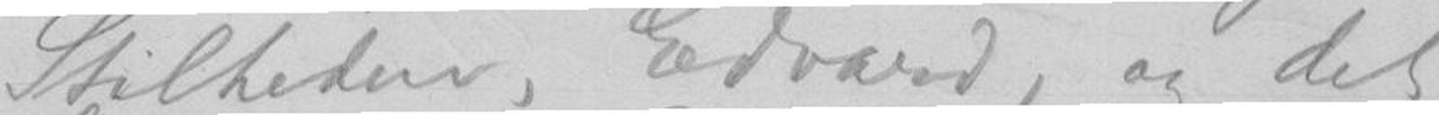Stilheden, Edvard, og det
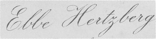Ebbe Hertzberg
